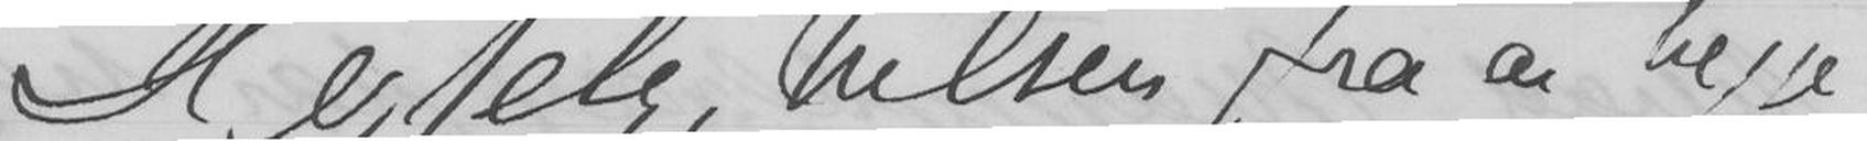Hjertelig, hilsen fra os begge
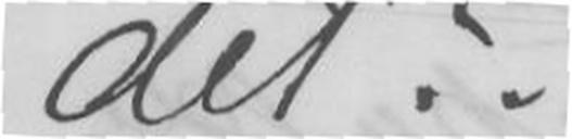det?!
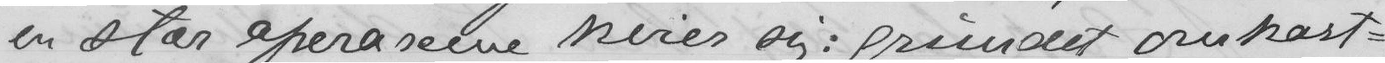en stor operascene kvier sig: grundet omkost-
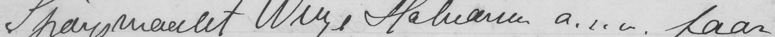Spørgsmaalet Winge Halvorsen o.s.v. faar
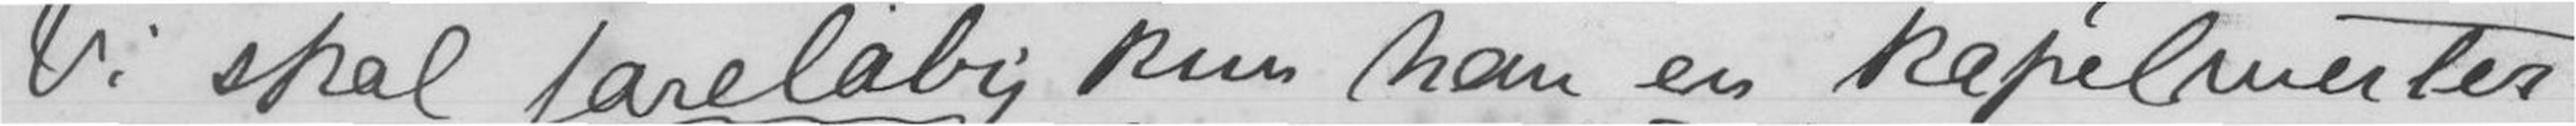Vi skal foreløbig kun have en kapelmester.
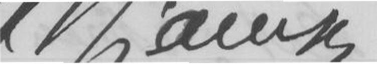B. Bjørnson
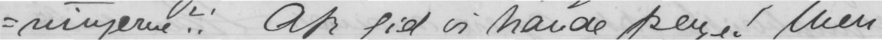-ningerne?! Ak gid vi havde penge! Men
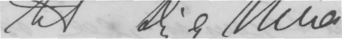til Dig og Nina
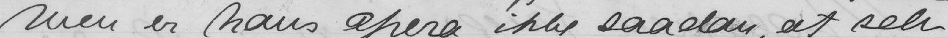Men er hans opera ikke saadan, at selv
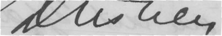Din Ven
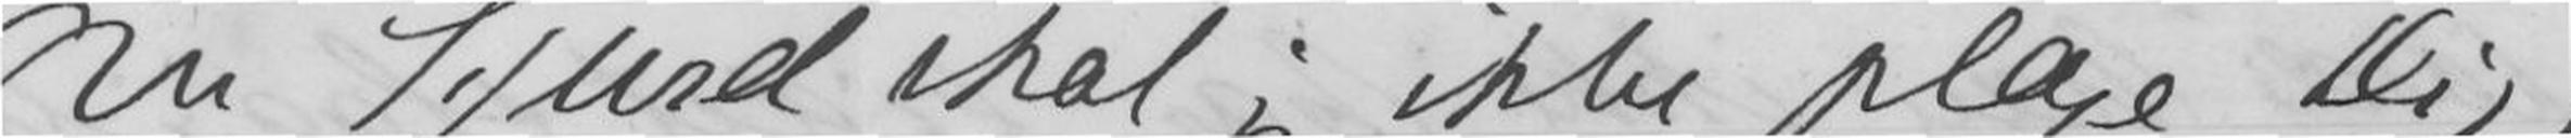Om Sigurd skal jeg ikke plage Dig;
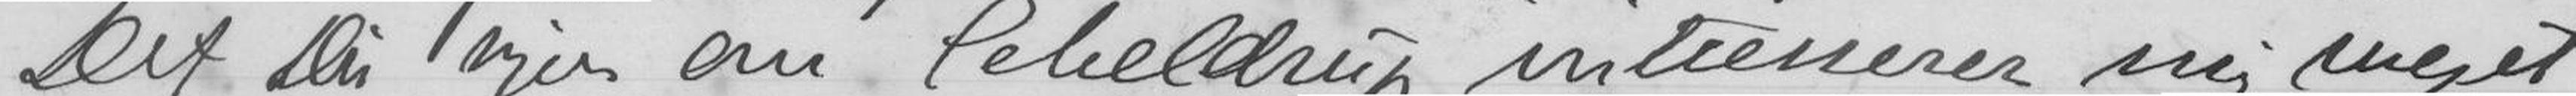Det Du siger om Scheldrup intresserer mig meget.
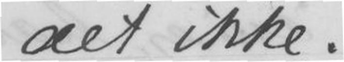det ikke!
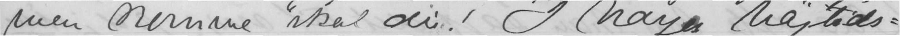men komme skal du! I Norges højtids-
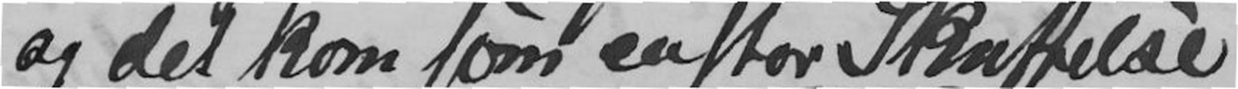og det kom som en stor Skuffelse
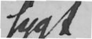sygt
